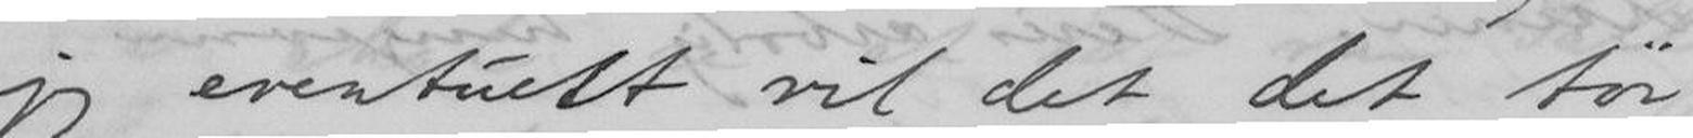jeg eventuelt vil det, det tør
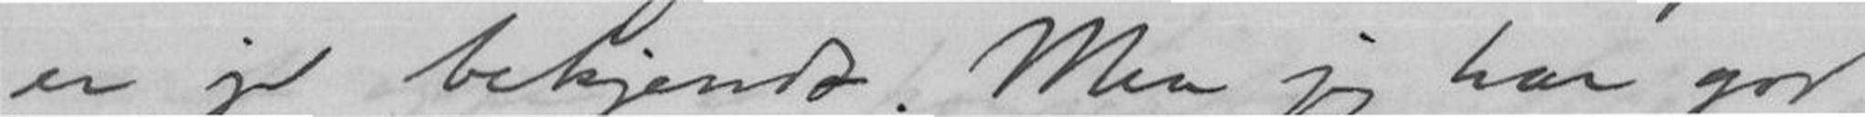er jo bekjendt. Men jeg har god
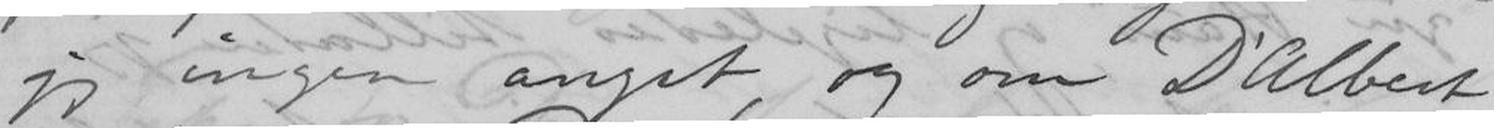jeg ingen angst, og om D'Albert
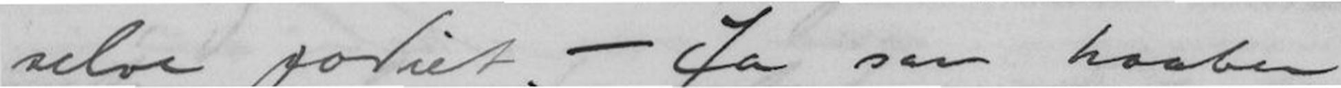selve podiet. - Ja saa haaber
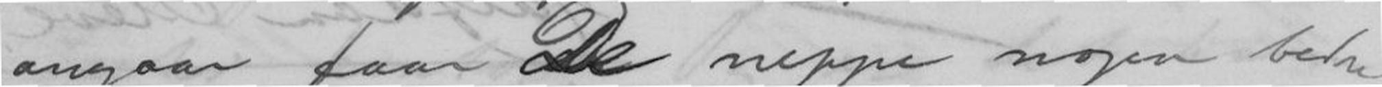angaar faar De neppe nogen bedre
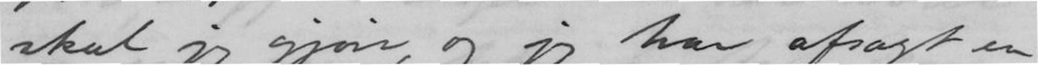skal jeg gjøre, og jeg har afsagt en
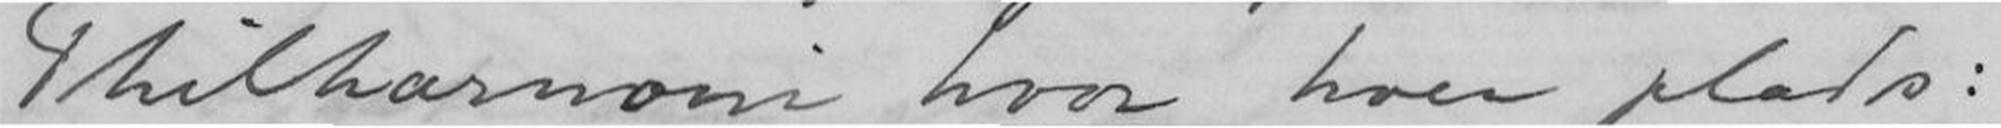Philharmoni hvor hver plads:
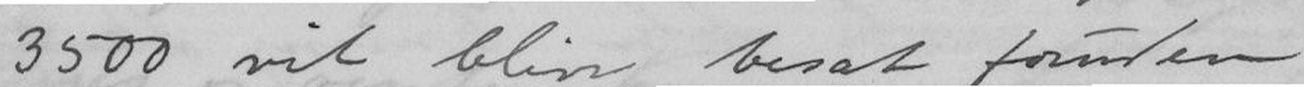3500 vil blive besat foruden
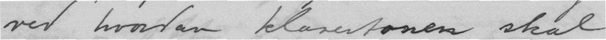ved hvordan klavertonen skal
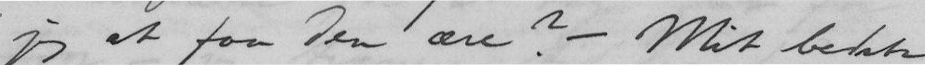jeg at faa den ære? - Mit bedste
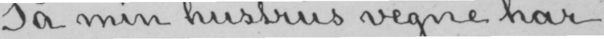På min hustrus vegne har
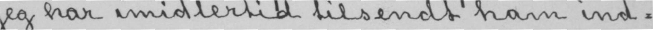Jeg har imidlertid tilsendt ham ind-
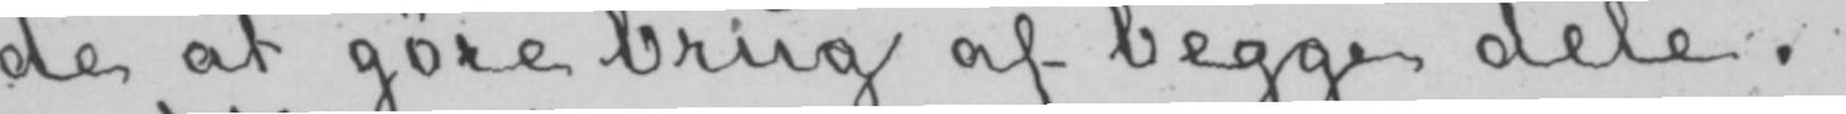de at gøre brug af begge dele.
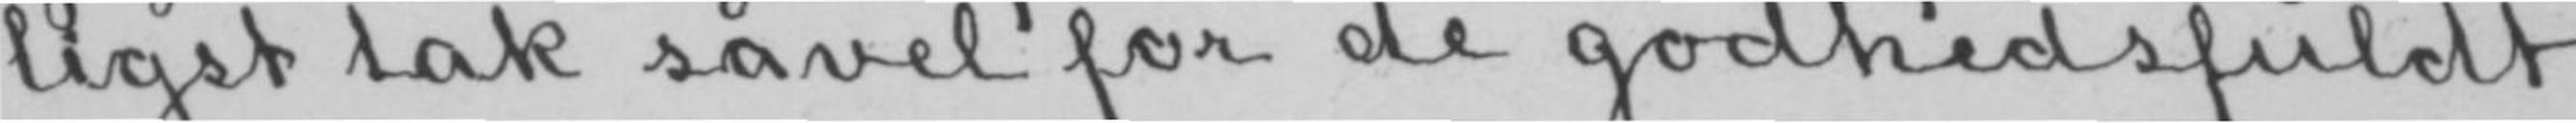ligst tak såvel for de godhedsfuldt
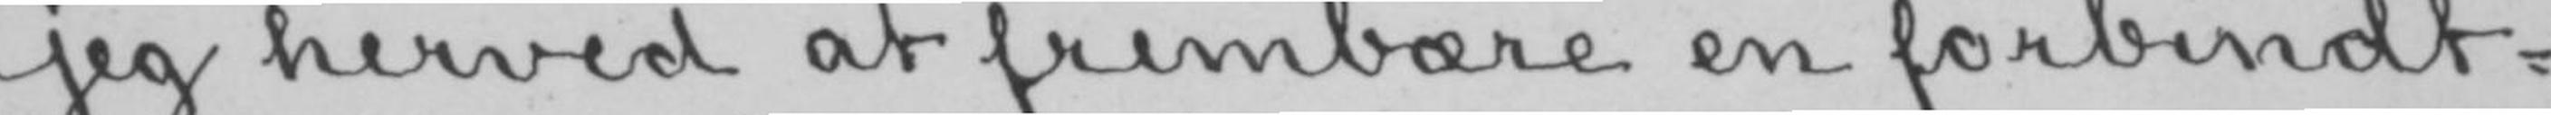jeg herved at frembære en forbindt-
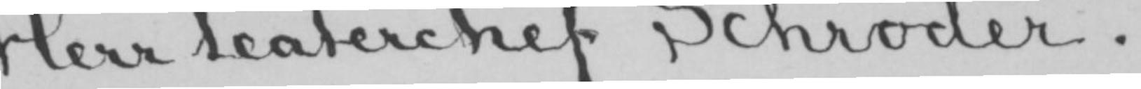Herr teaterchef Schrøder.
